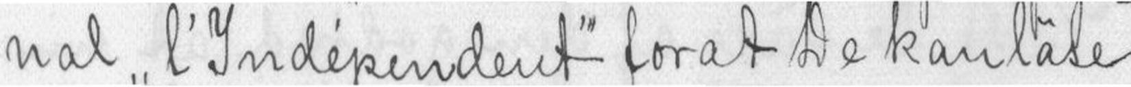nal l'Indépendent forat De kan läse
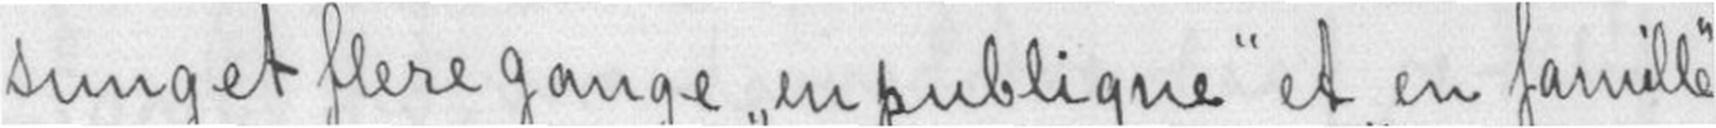sunget flere gange en publique et en famille
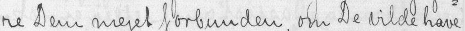re Dem meget forbunden, om De vilde have
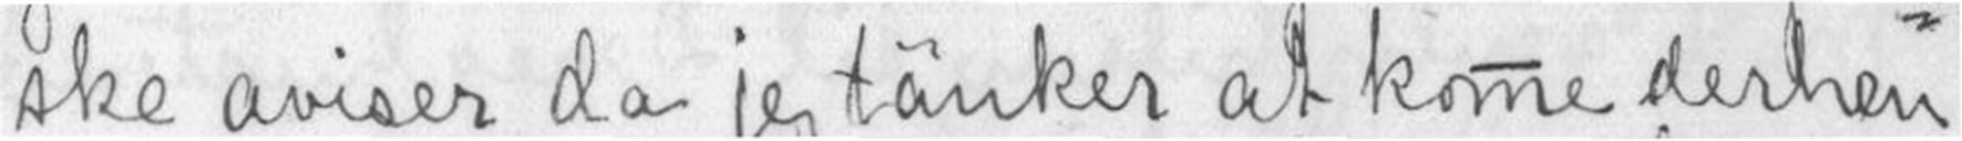ske aviser, da jeg tänker at komme derhen
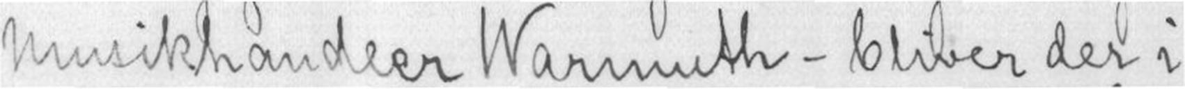musikhadler Warmuth - bliver der i
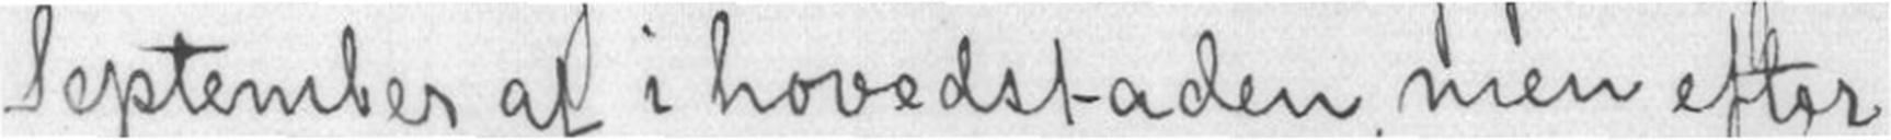September af i hovedstaden, men efter
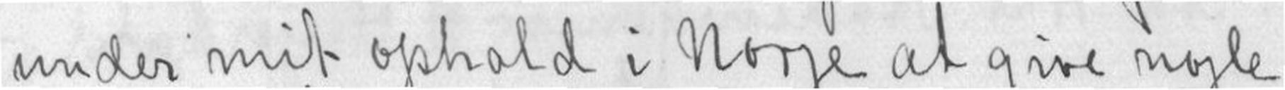under mit ophold i Norge at give nogle
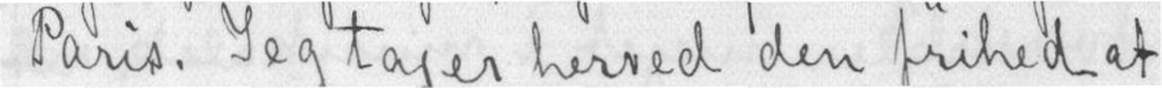i Paris. Jeg tager herved den frihed at
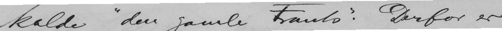kalde "den gamle Frants". Derfor er
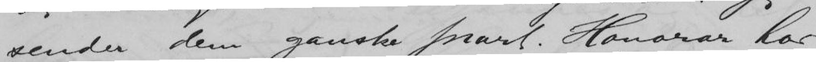sender dem ganske snart. Honorar har
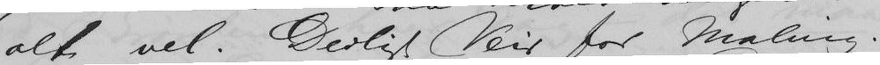alt vel. Deiligt Veir for Maling.
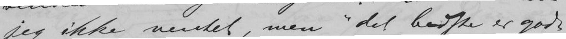jeg ikke ventet, men "det bedste er godt
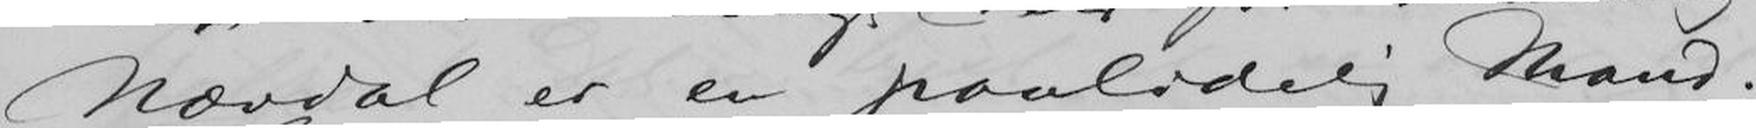Nævdal er en paalidelig Mand.
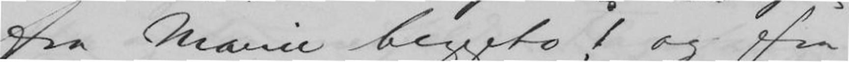fra Marie beggeto! og fra
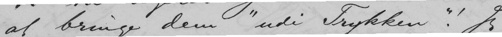at bringe dem "udi Trykken"! Jeg
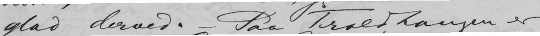glad derved. - Paa Troldhaugen er
